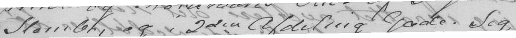Slembe, og i 2den Afdeling Gade. Jeg
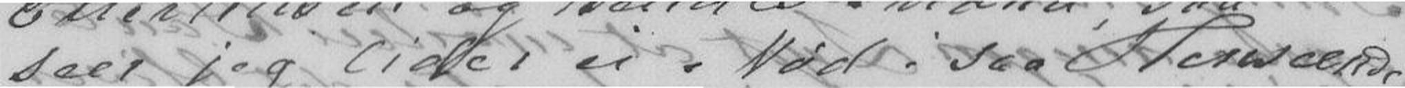seer jeg lider ei Nød i saa Henseende.
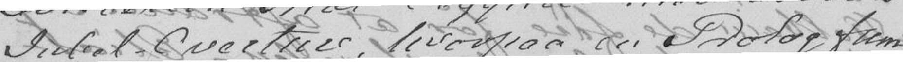Jubel-Overture, hvorpaa en Prolog frem
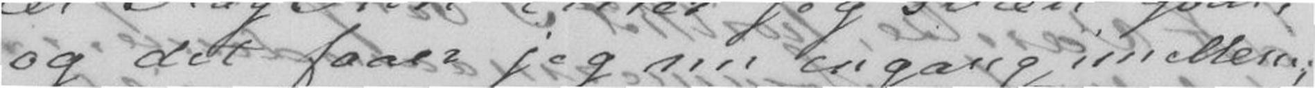og det faaer jeg nu engang imellem;
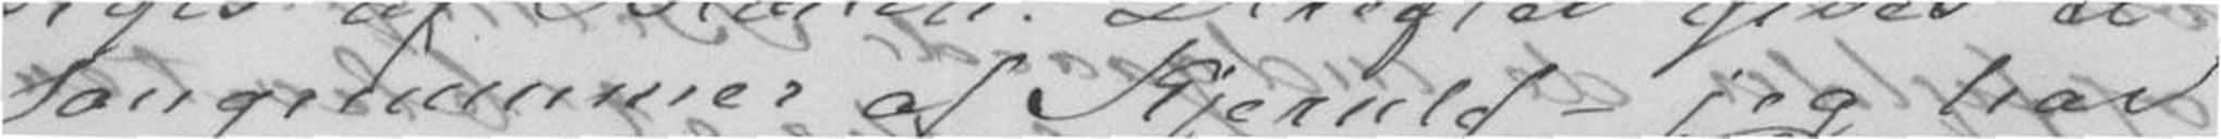Sangnummer af Kjerulf - jeg har
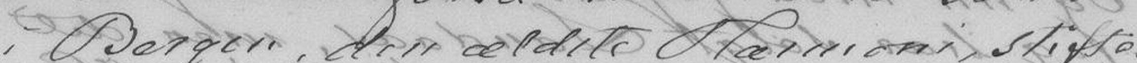i Bergen, den ældste Harmoni, stifte-
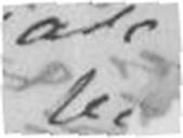bo
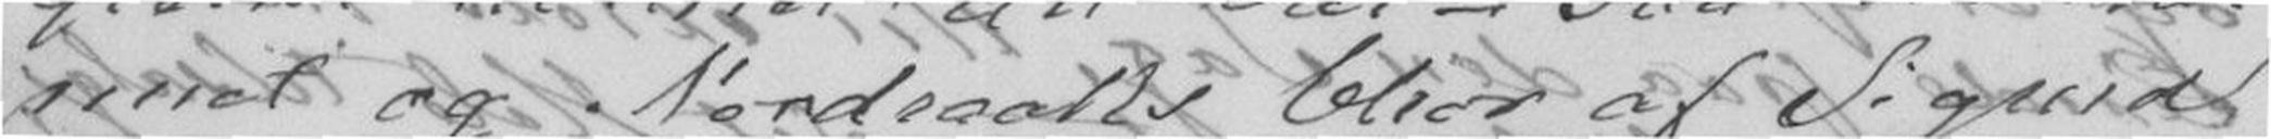nuet og Nordraaks Chor af Sigurd
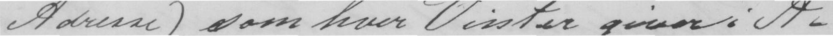Adresse) som hver Vinter giver i A-
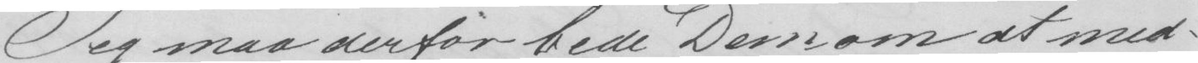Jeg maa derfor bede Dem om at med-
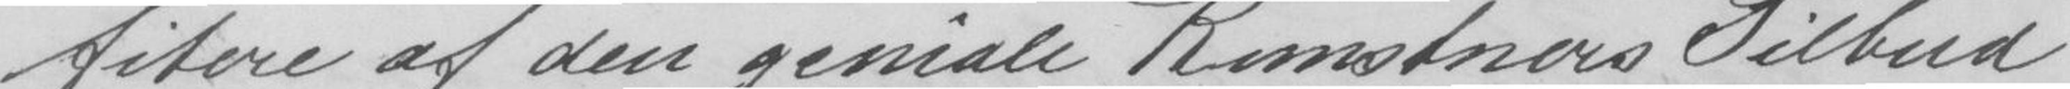fitere af den geniale Kunstners Tilbud,
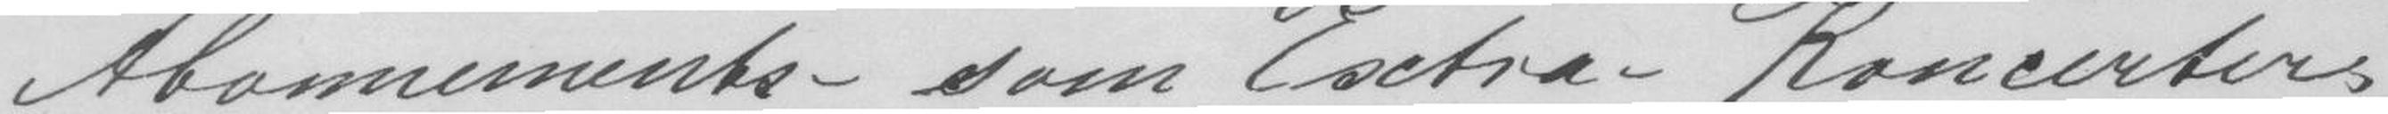Abonnements- som Extra-Koncerter.
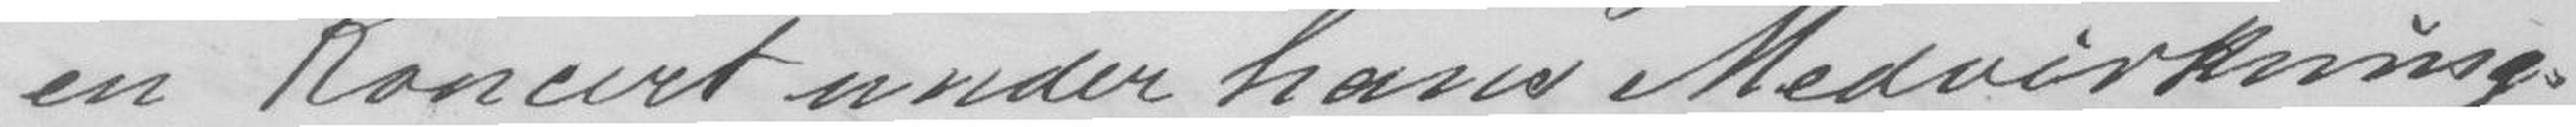en Koncert under hans Medvirkning.
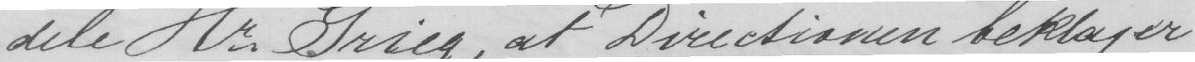dele Hr.Grieg, at Directionen beklager
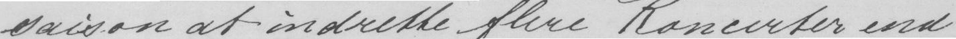saison at indrette flere Koncerter end
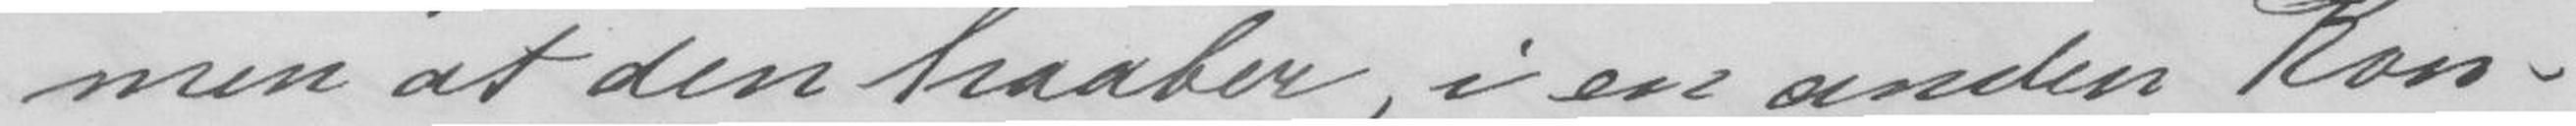men at den haaber, i en anden Kon-
